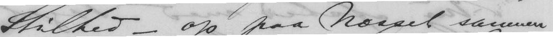Stilhed - op paa Næsset sammen
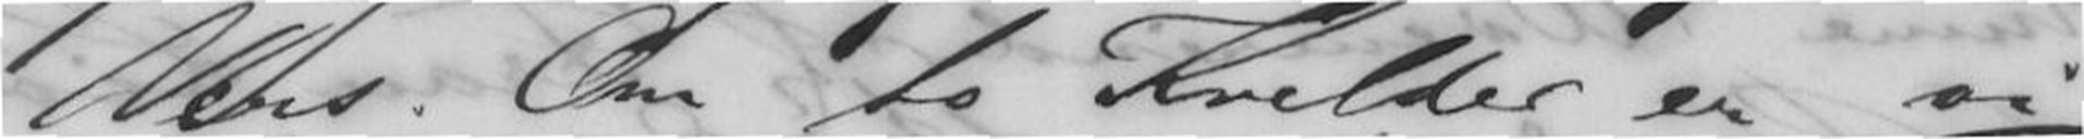Vers. Om to Kvelder er vi
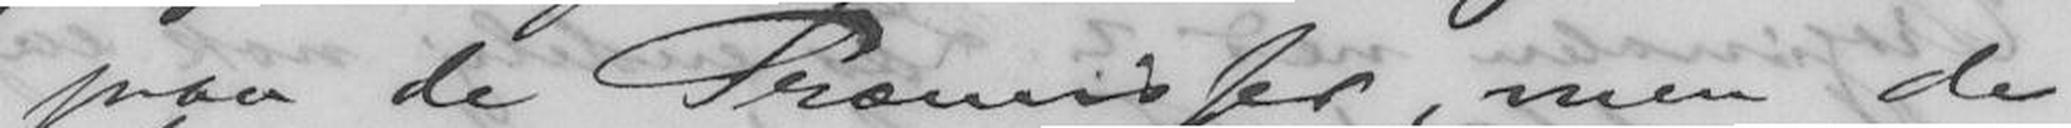paa de Præmisser, men de
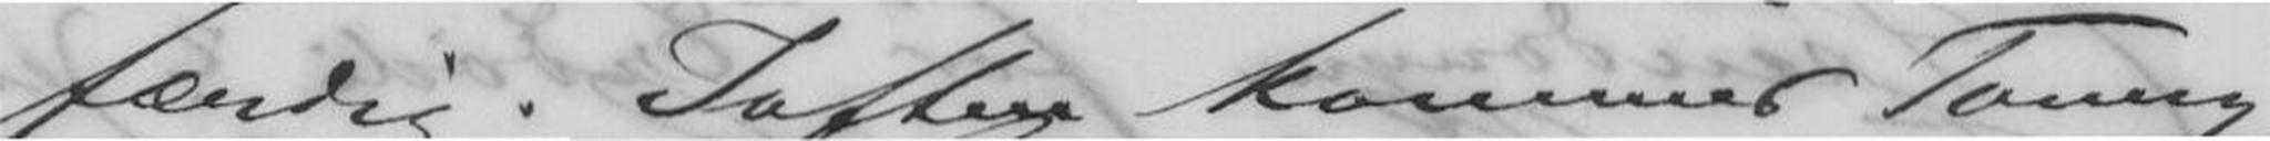færdig. Iaften kommer Tonny
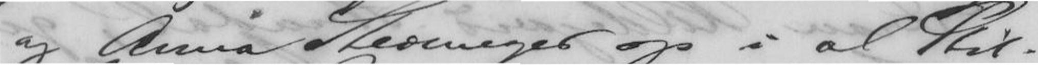og Anna Steomeyer op i al Stil-
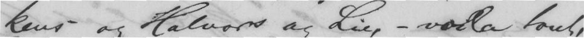kens og Halvors og Lie, - voila tout!
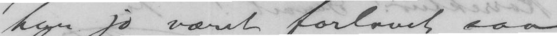har jo været forlovet saa
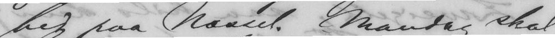hed paa Næsset. Mandag skal
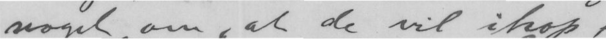noget om at de vil ihop,
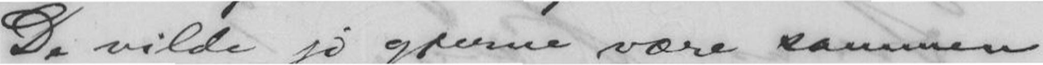De vilde jo gjerne være sammen
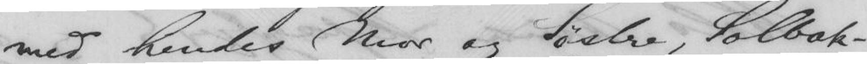med hendes Mor og Søstre, Solbak-
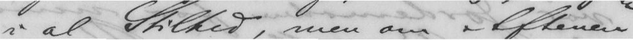i al stilhed, men om Aftenen
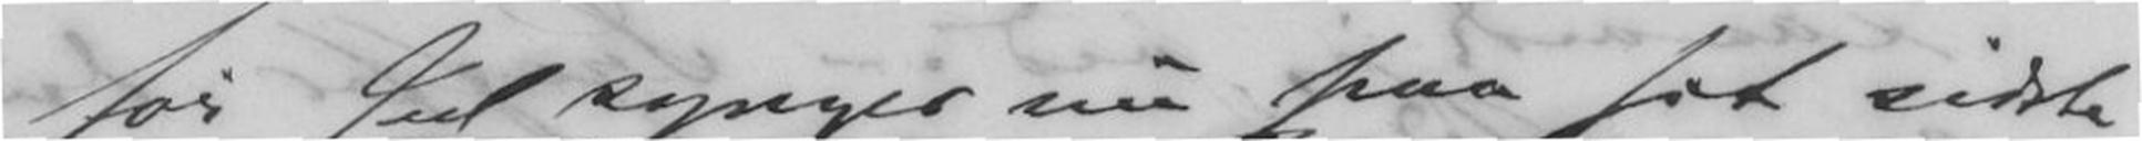før Jul synger nu paa sit sidste
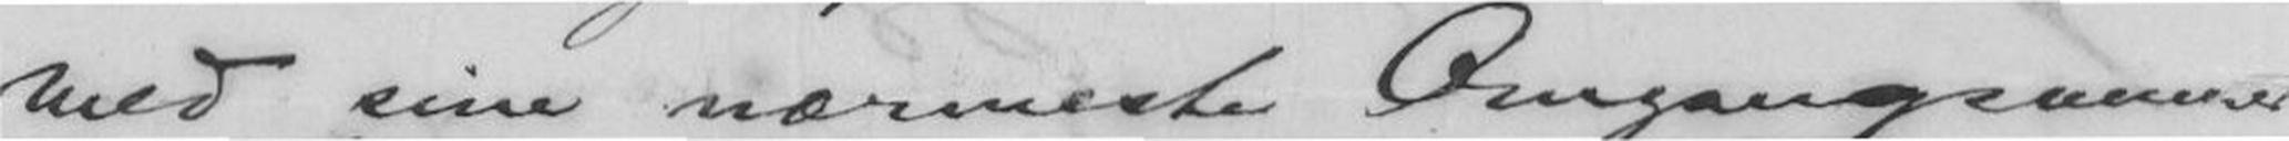med sine nærmeste Omgangsvenner
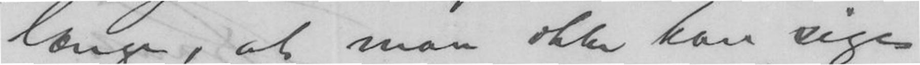længe, at man ikke kan sige
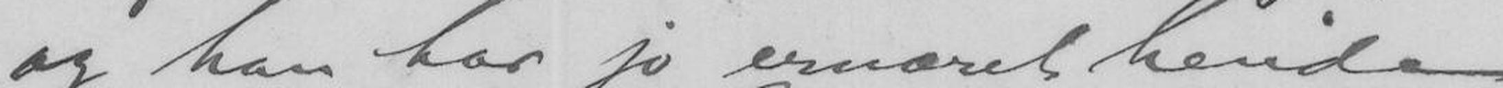og han har jo ernæret hende
